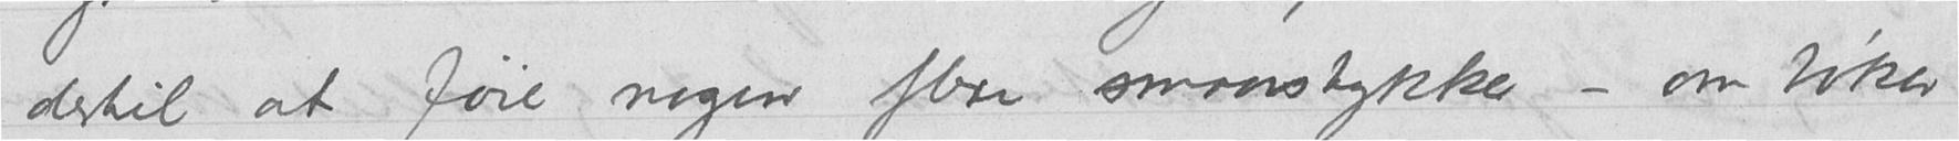dertil at føie nogen flere smaastykker – om bøker
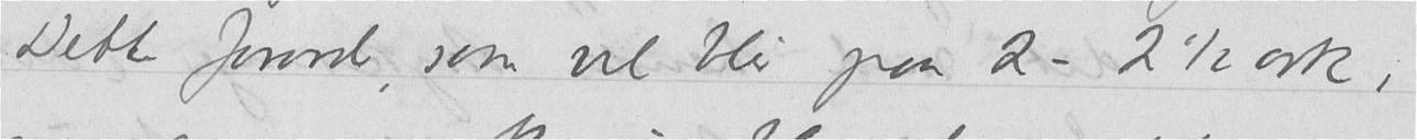Dette forord, som vil blir paa 2 - 2 ½ ark,
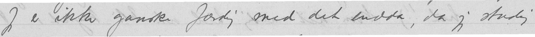Jeg er ikke ganske færdig med det endda, da jeg stadig
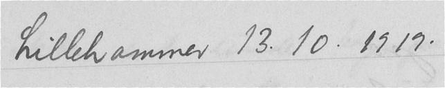Lillehammer 13.10.1919.
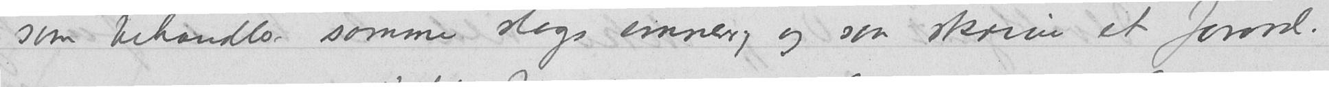som behandler samme slags emner, og saa skrive et forord.
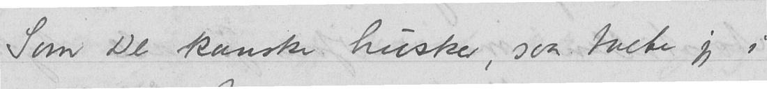Som De kanske husker, saa talte jeg i
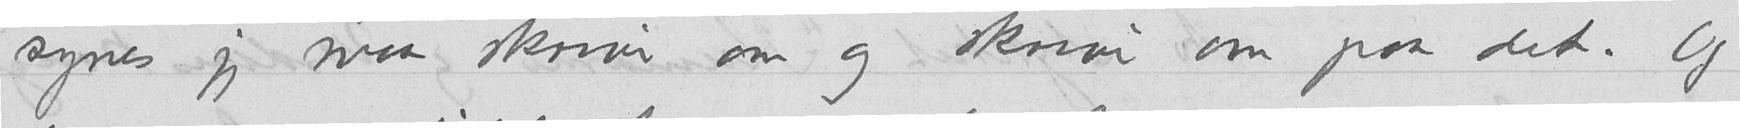synes jeg maa skrive om og skrive om paa det. Og
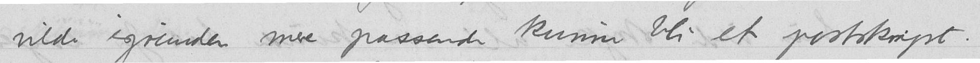vilde igrunden mere passende kunne bli et postskript.
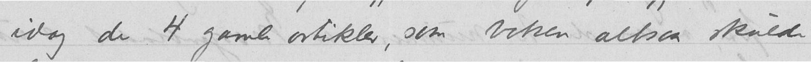idag de 4 gamle artikler, som boken altsaa skulde
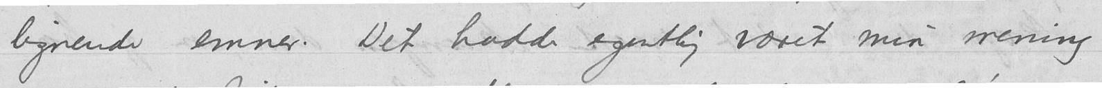lignende emner. Det hadde egentlig været min mening
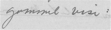gammel vise:
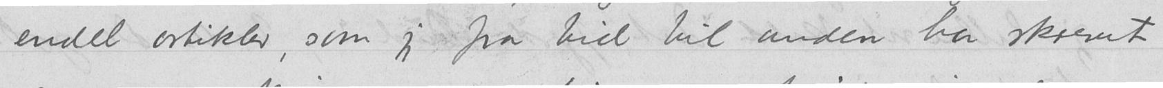endel artikler, som jeg fra tid til anden har skrevet
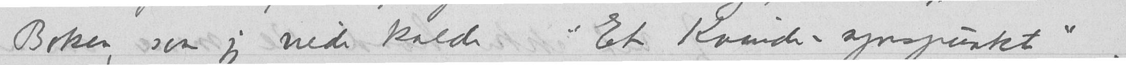Boken, som jeg vilde kalde «Et Kvinde-synspunkt»,
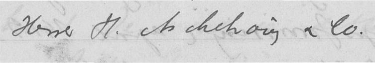Herrer H. Aschehaug & Co.
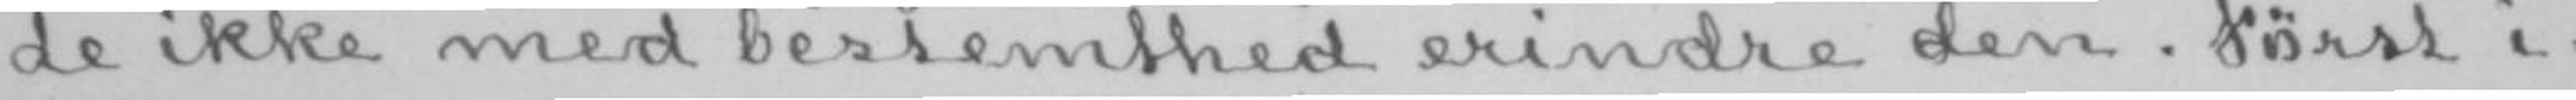de ikke med bestemthed erindre den. Først i-
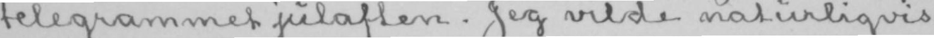telegrammet julaften. Jeg vilde naturligvis
